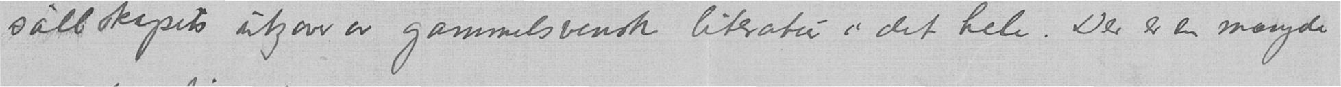sällskapets utgaver av gammelsvensk literatur i det hele. Der er en mængde
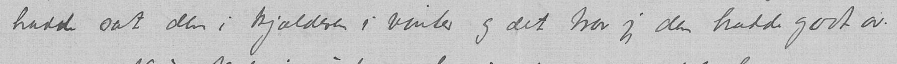hadde sat den i kjælderen i vinter og det tror jeg den hadde godt av.
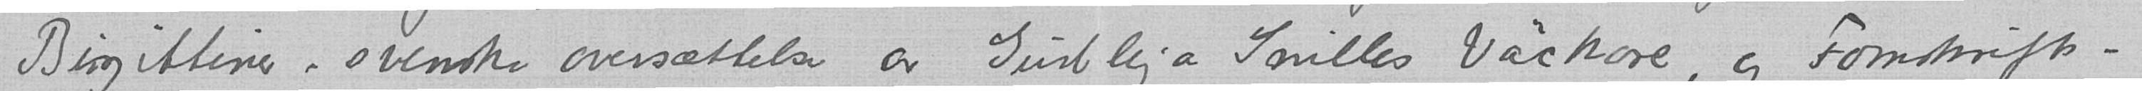Birgittiner-svenske oversættelse av Gudeliga Snilles Väckare, og Formskrifts-
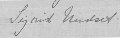Sigrid Undset.
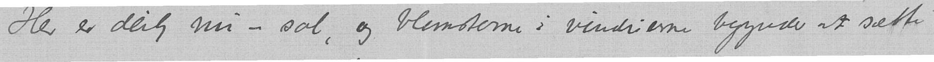Her er deilig nu - sol, og blomsterne i vinduerne begynder at sætte
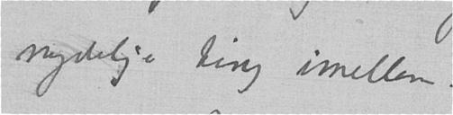nydelige ting imellem.
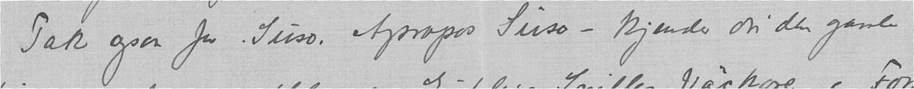Tak ogsaa for Suso. Apropos Suso – kjender du den gamle
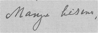Mange hilsener,
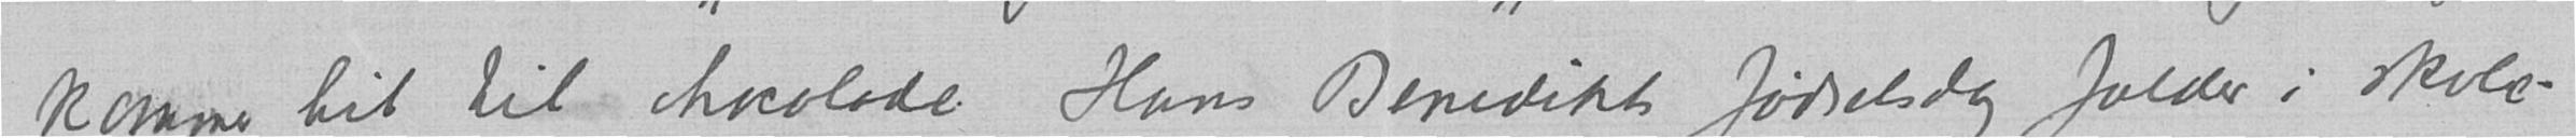kommer hit til chocolade. Hans Benedikts fødselsdag falder i skole-
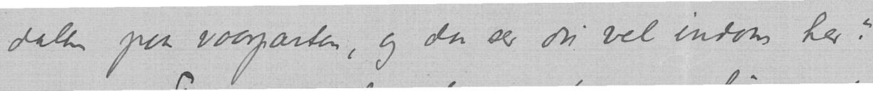dalen paa vaarparten, og da ser du vel indom her?
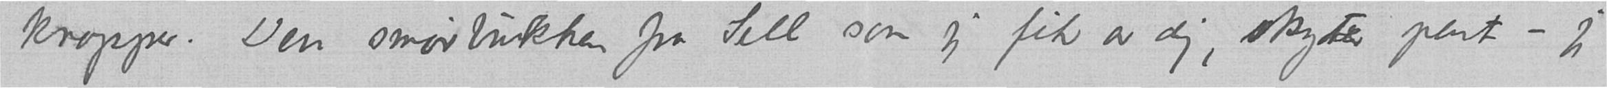knopper. Den smørbukken fra Sell som jeg fik av dig, skyter pent – jeg
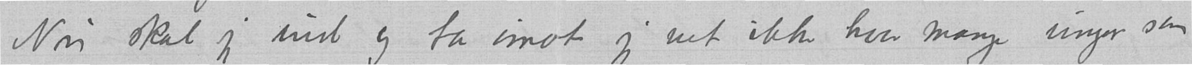Nu skal jeg ind og ta imot jeg vet ikke hvor mange unger som
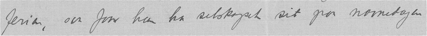ferien, saa faar han ha selskapet sit paa navnedagen.
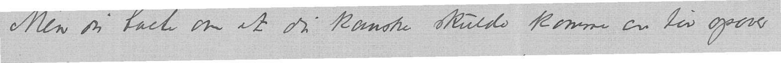Men du talte om at du kanske skulde komme en tur opover
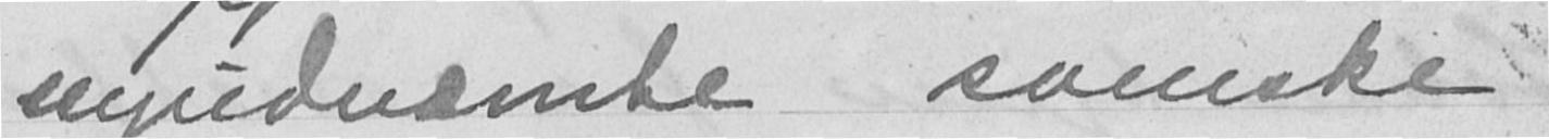nyudnævnte svenske
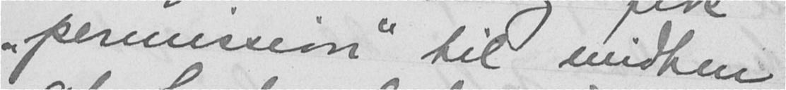"permission" til midten
